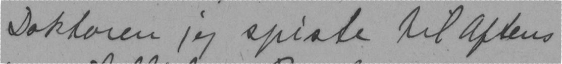Doktoren jeg spiste til aftens
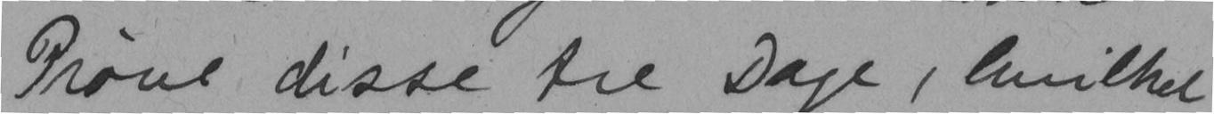Prøve disse tre Dage, hvilket
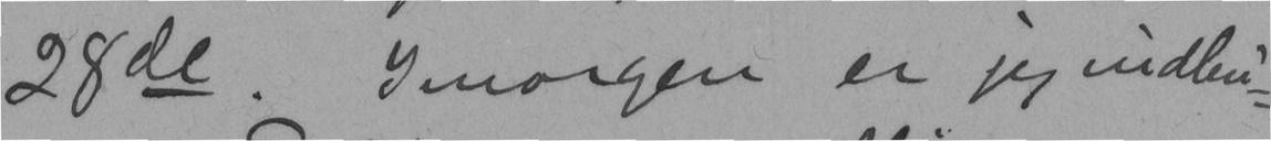28de. Imorgen er jeg indleu-
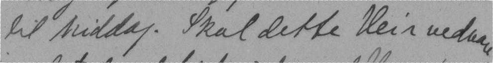til middag. Skal dette Veir vedvare
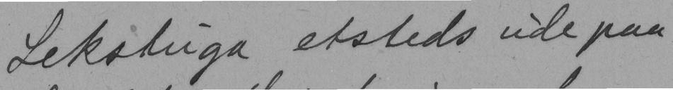Lekstuga etsteds ude paa
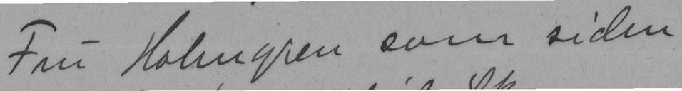Fru Holmgren som siden
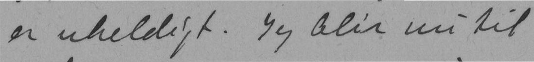er uheldigt. Jeg blir nu til
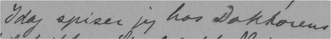Idag spiser jeg hos Doktorens
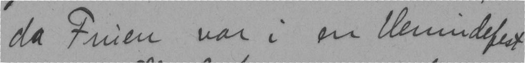da Fruen var i en venindefest
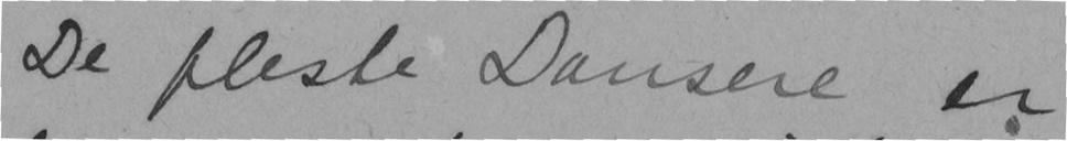De fleste Dansere er
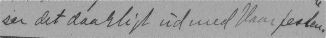ser det daarligt ud med Vaarfesten.
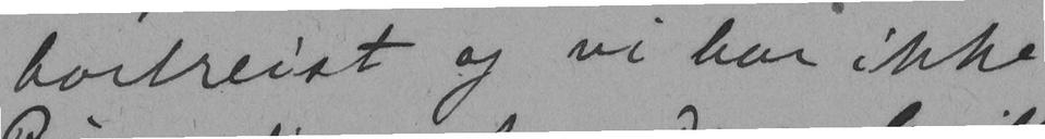bortreist og vi har ikke
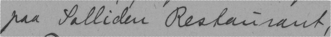paa Solliden Restaurant,
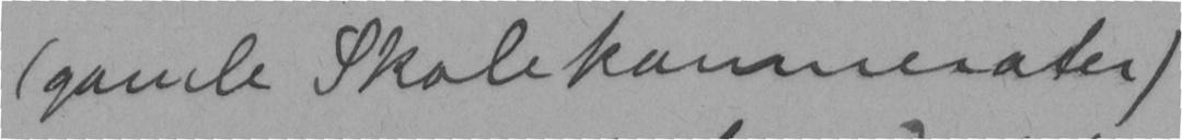(gamle Skolekammerater)
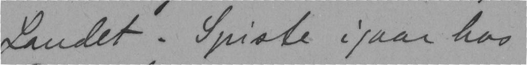Landet. Spiste igaar hos
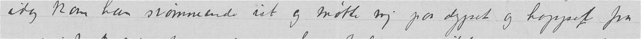idag kom han svømmende ut og møtte mig paa dypet og hoppet fra
